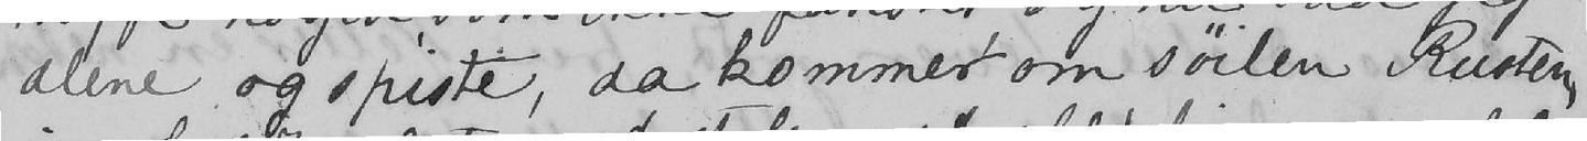alene og spiste, da kommer om søilen Rusten,
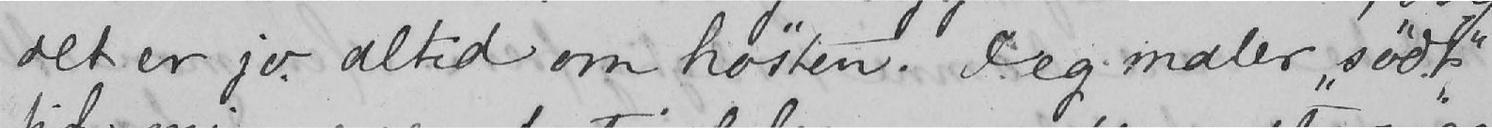det er jo altid om høsten. Jeg maler «sødt»
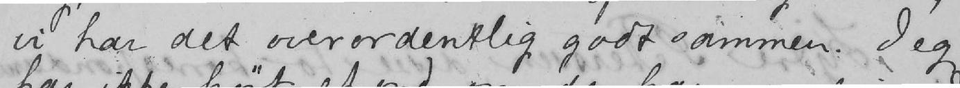vi har det overordentlig godt sammen. Jeg
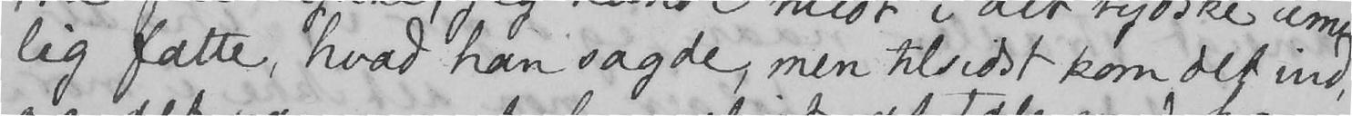lig fatte, hvad han sagde, men tilsidst kom det ind,
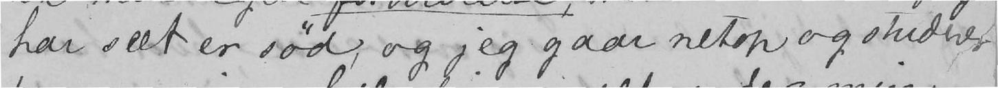har seet er sød, og jeg gaar netop og studerer
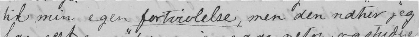til min egen fortvivlelse, men den natur jeg
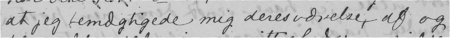at jeg bemægtigede mig deres værelse, af og
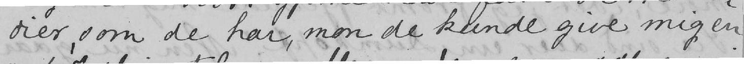dier, som de har, mon de kunde give mig en
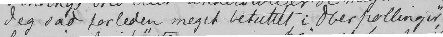Jeg sad forleden meget betuttet i «Oberpollinger»,
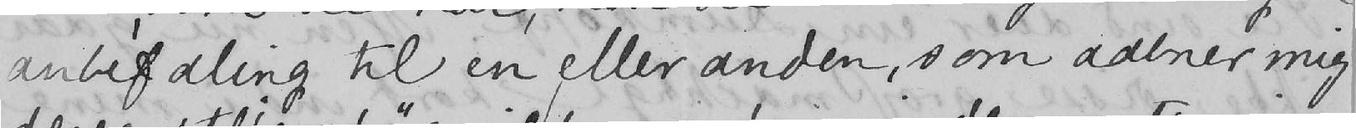anbefaling til en eller anden, som aabner mig
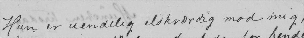Hun er uendelig elskværdig mod mig,
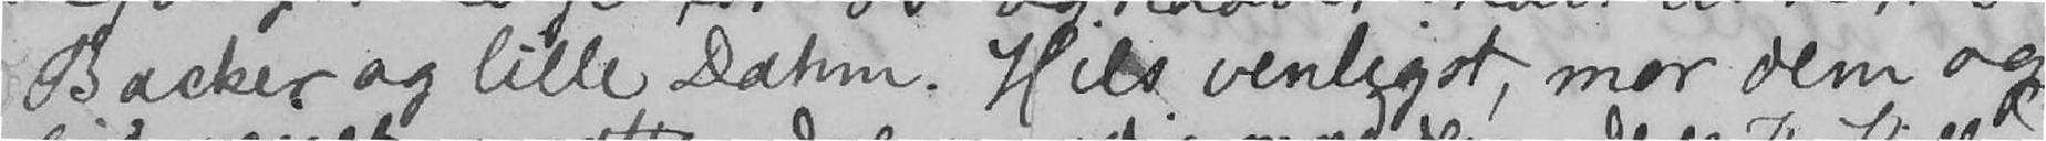Backer og lille Dahm. Hils venligst, mor dem og
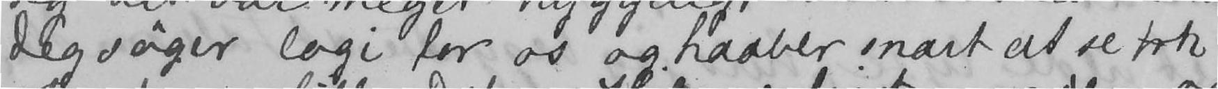Jeg søger logi for os og haaber snart at se frk
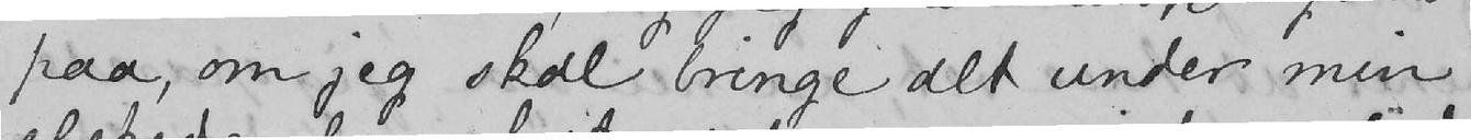paa, om jeg skal bringe alt under min
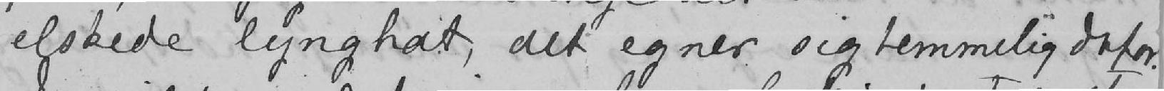elskede lynghat, det egner sig temmelig derfor.
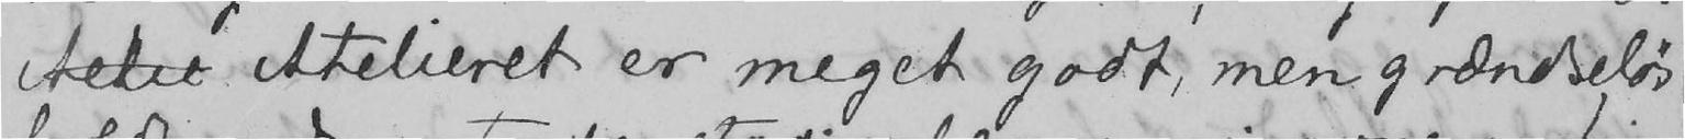Aetel Atelieret er meget godt, men grændseløs
